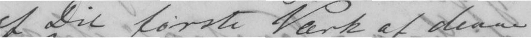af Dit første Værk af denne
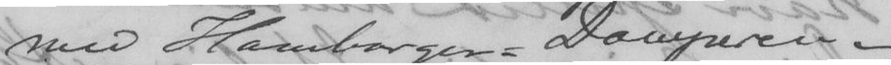med Hamborger-Damperen.
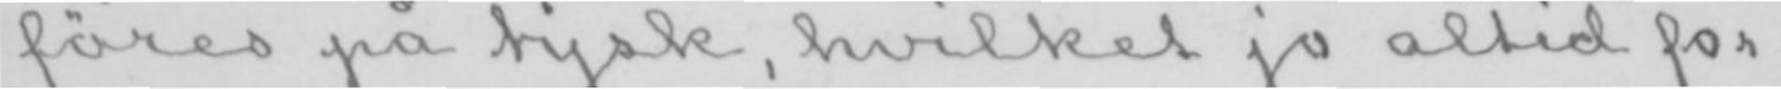føres på tysk, hvilket jo altid for-
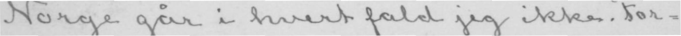Norge går i hvert fald jeg ikke. For-
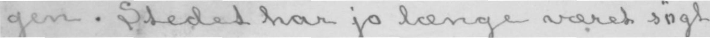gen. Stedet har jo længe været søgt
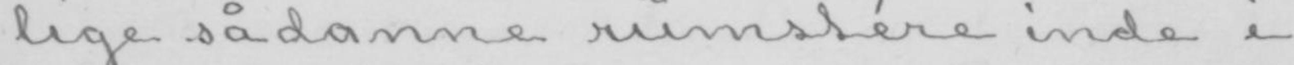lige sådanne rumstére inde i
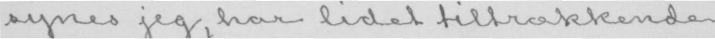synes jeg, har lidet tiltrækkende
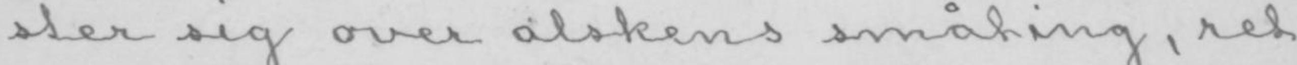ster sig over alskens småting, ret
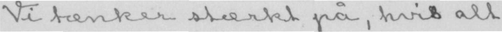Vi tænker stærkt på, hvis alt
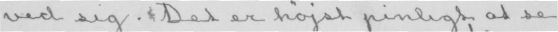ved sig. Det er højst pinligt at se
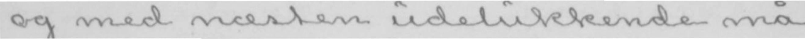og med næsten udelukkende må
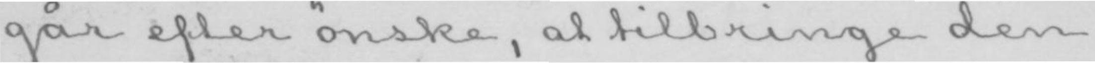går efter ønske, at tilbringe den
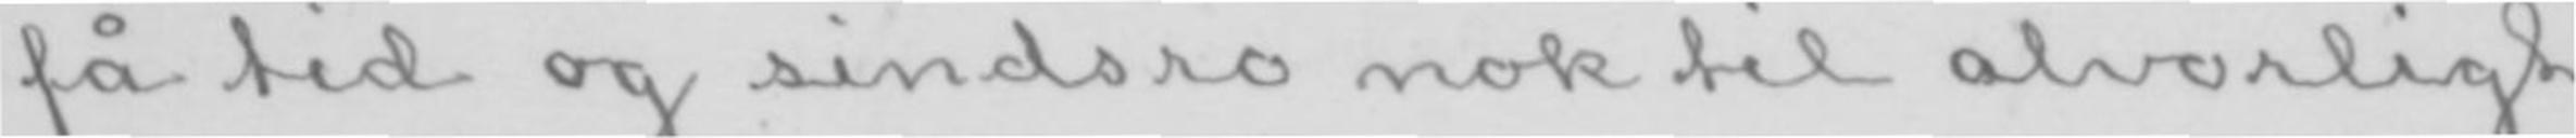få tid og sindsro nok til alvorligt
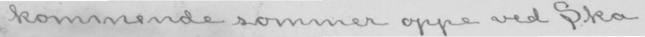kommende sommer oppe ved Ska-
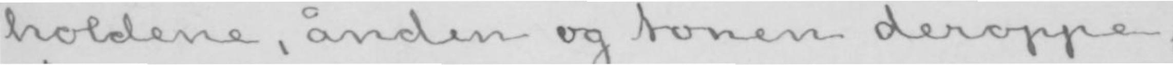holdene, ånden og tonen deroppe,
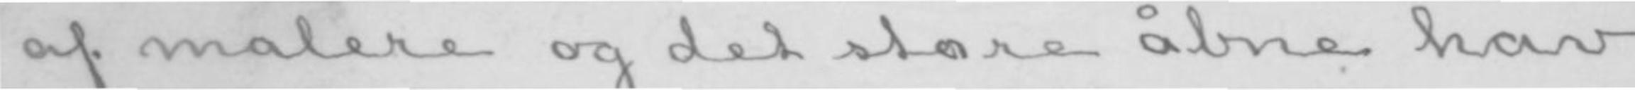af malere og det store åbne hav
